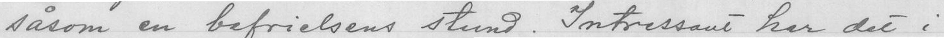såsom en befrielsens stund. Intressant har det i
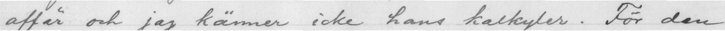affär och jag känner icke hans kalkyler. För den
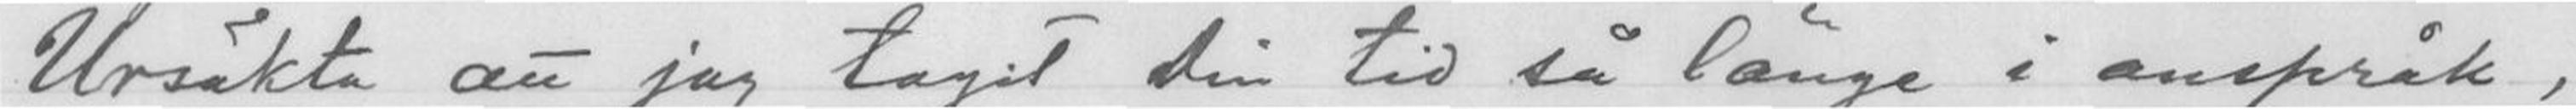Ursäkta att jag tagit Din tid så länge i anspråk,
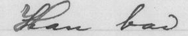Han bad
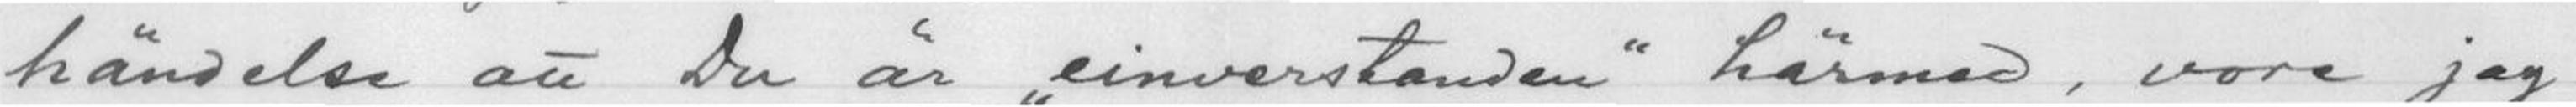händelse att Du är einverstanden härmed, vore jag
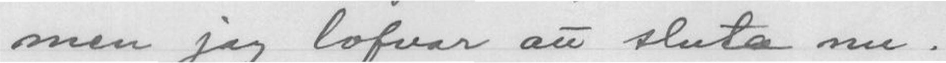men jag lofvar att sluta nu.
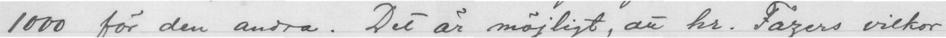1000 för den andra. Det är möjligt, att hr. Fagers vilkor
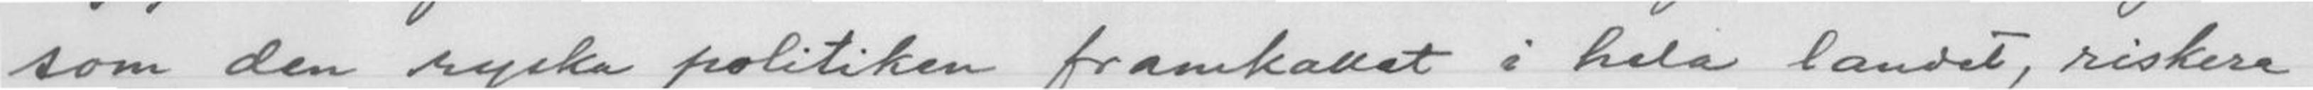som den rycka politiken framkallat i hela landet, riskera
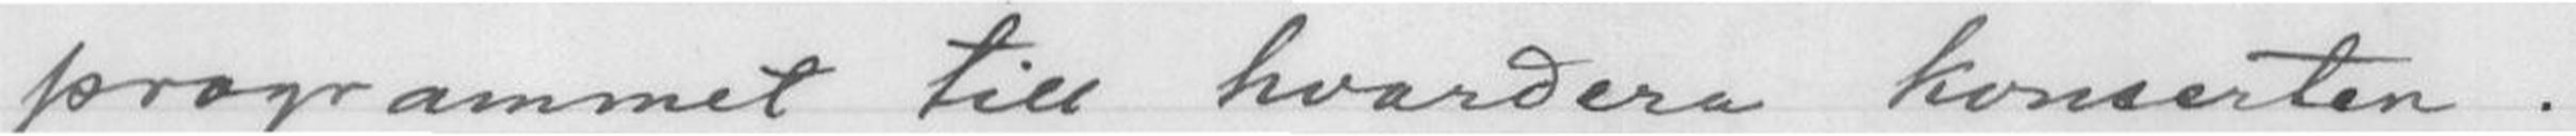programmet till hvardera konserten.
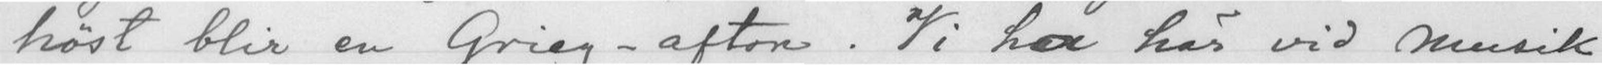höst blir en Grieg - afton. Vi ha här vid musik
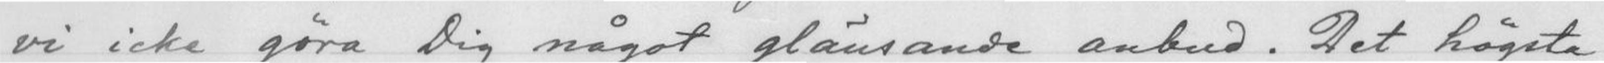vi icke göra Dig något glänsande anbud. Det högsta
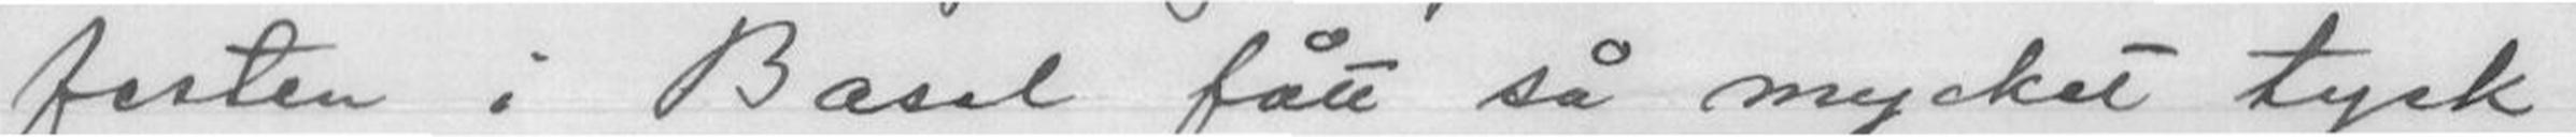festen i Basil fått så mycket tysk
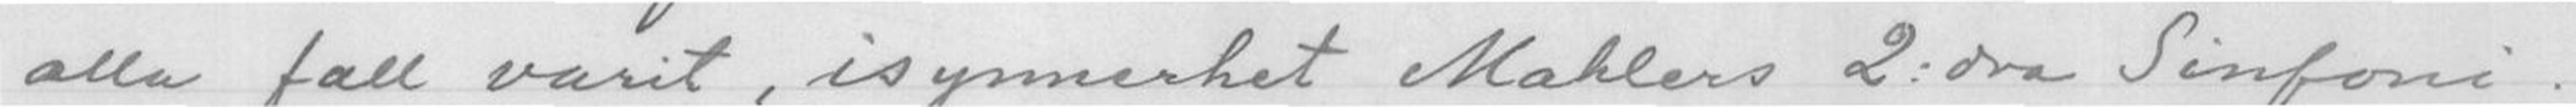alla fall varit, isymmerhet mahlers 2:dra Sinfoni.
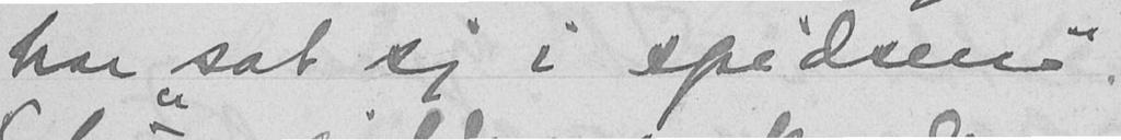har sat sig i spidsen
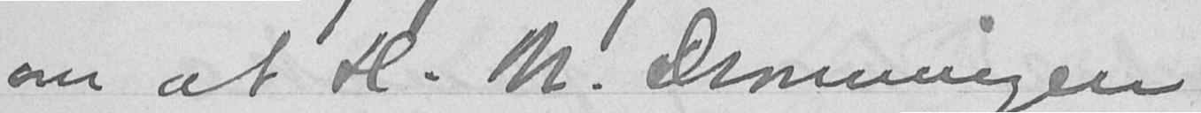om at H. M. Dronningen
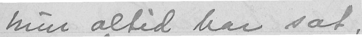hun altid har sat,
